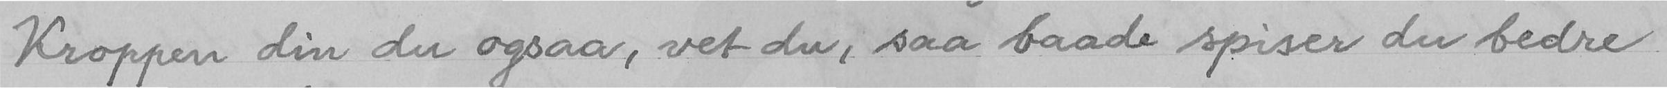Kroppen din du ogsaa, vet du, saa baade spiser du bedre
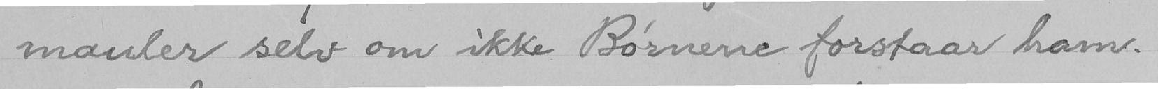mauler selv om ikke Børnene forstaar ham.
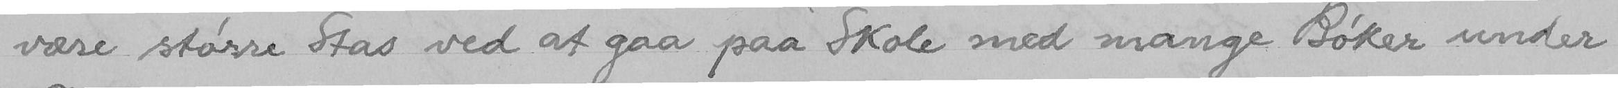være større Stas ved at gaa paa Skole med mange Bøker under
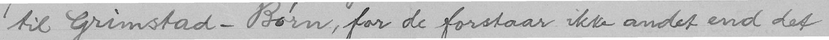til Grimstad-Børn, for de forstaar ikke andet end det
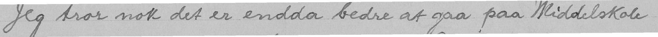Jeg tror nok det er endda bedre at gaa paa Middelskole
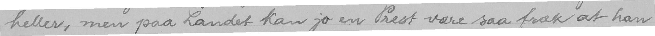heller, men paa Landet kan jo en Prest være saa fræk at han
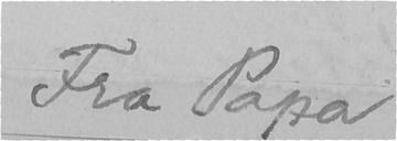Fra Papa
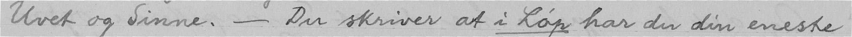Uvet og Sinne. - Du skriver at i Løp har du din eneste
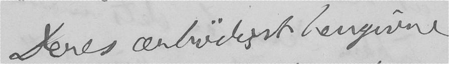Deres ærbødigst hengivne
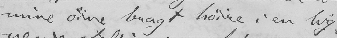mine øine bragt høire i en lig-
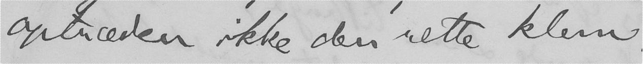optræden ikke den rette klem.
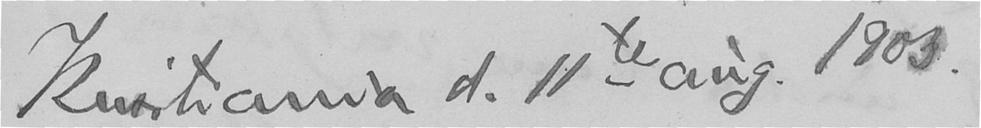Kristiania d. 11te aug. 1903.
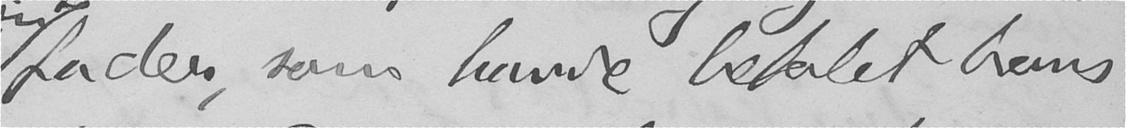fader, som havde befalet hans
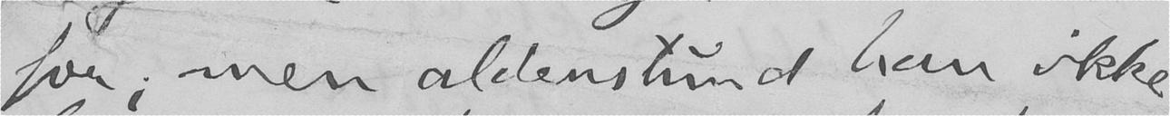for; men aldenstund han ikke
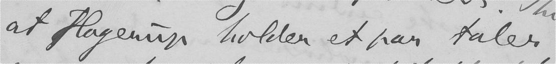at Hagerup holder et par taler,
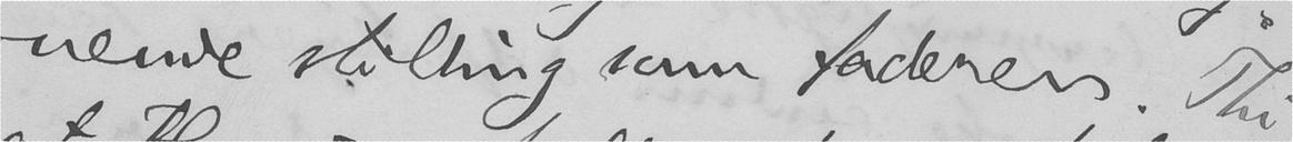nende stilling som faderen. Thi
